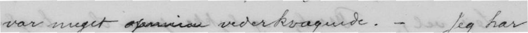var meget op...vederkvægende. - Jeg har
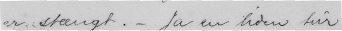er stængt. - Ja en liden tur
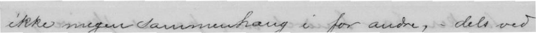ikke megen sammenheng i for andre, dels ved
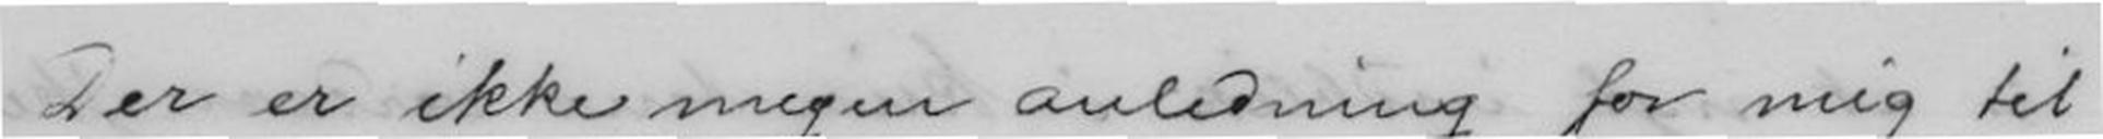Der er ikke megen andledning for mig til
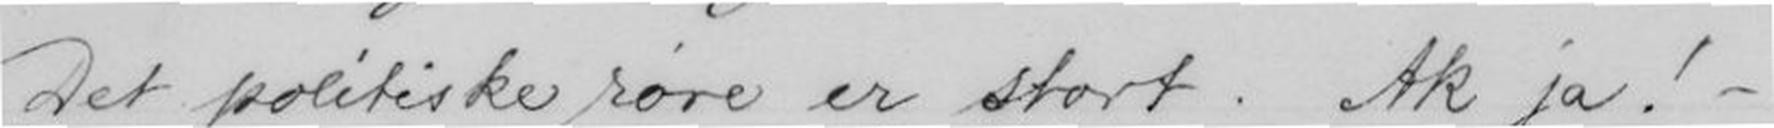Det politiske røre er stort. Ak ja! -
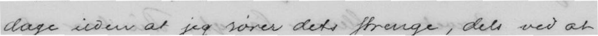dage uden at jeg rører dets strenge, dels ved at
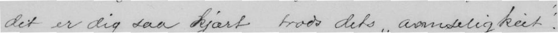det er dig saa kjært trods dets annseligkeit.
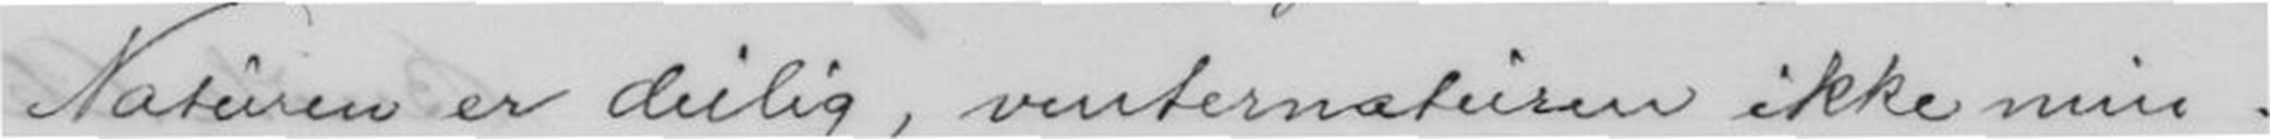Naturen er deilig, vinternaturen ikke min-
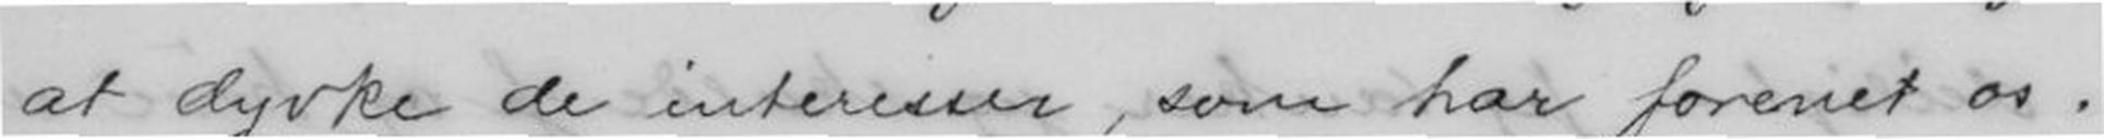at dyrke de interesser, som har forenet os.
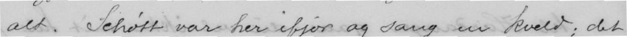alt. Schøtt var her ifjor og sang en kveld; det
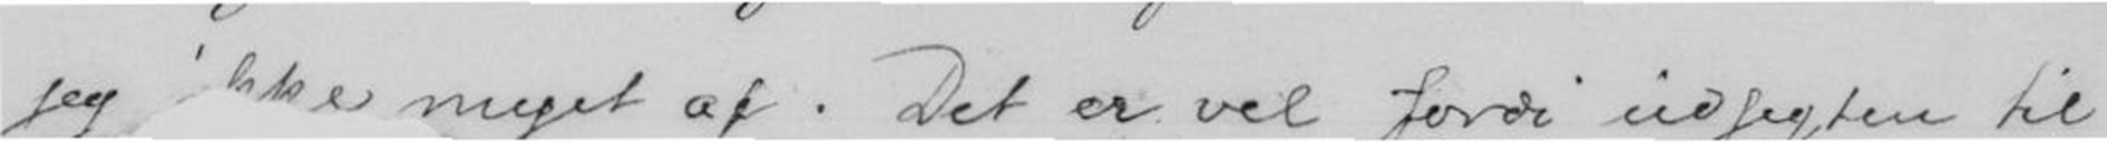jeg ikke meget af. Det er vel fordi udsigten til
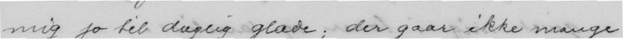mig jo til daglig glæde; der gaar ikke mangen
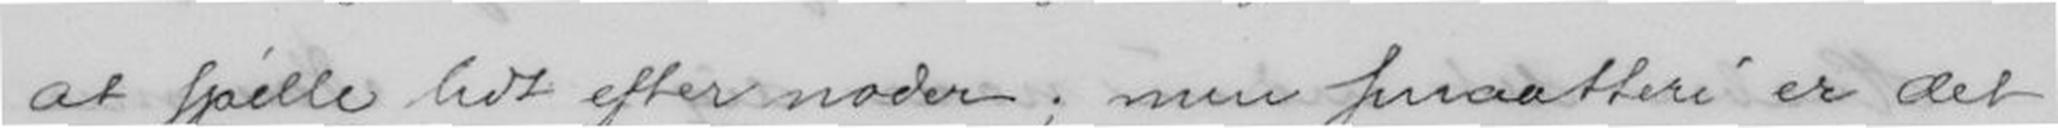at spille lidt efter noder; men smaateri er det
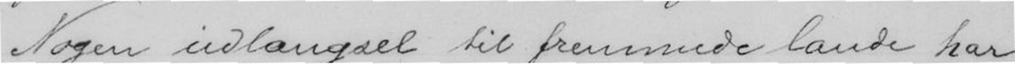Nogen udlængsel til fremmede lande har
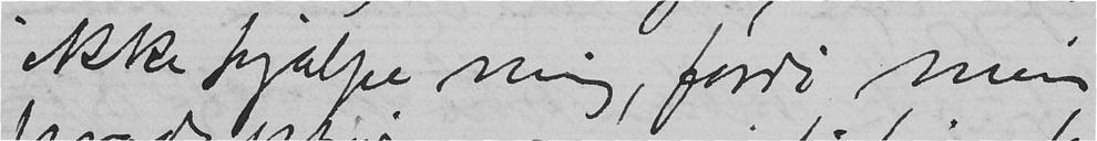ikke hjælpe mig, fordi min
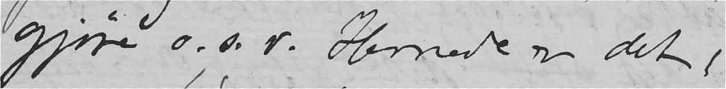gjøre o.s.v. Hernede er det,
